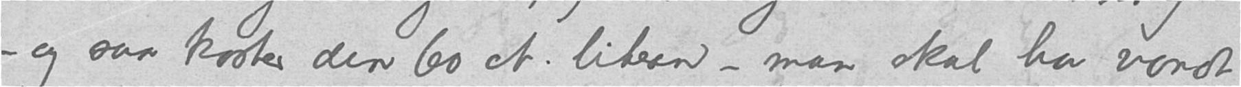– og saa koster den 60 ct. literen – man skal ha vondt
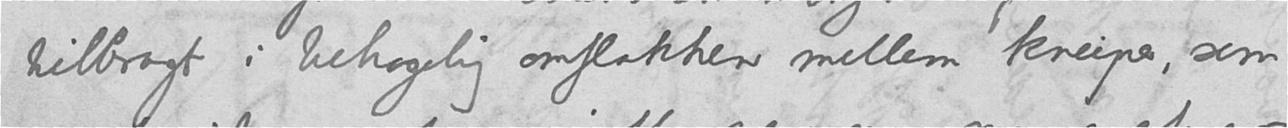tilbragt i behagelig omflakken mellem kneiper, som
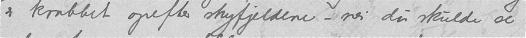er krabbet opefter skyfjeldene – nei du skulde se
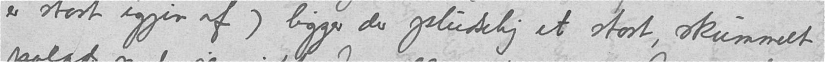er stort igjen af) ligger der pludselig et stort, skummelt
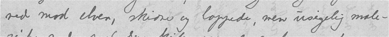ned mod elven, skidne og loppede, men usigelig male-
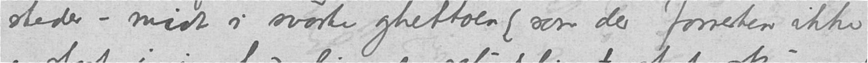steder – midt i svarte ghettoen (som der forresten ikke
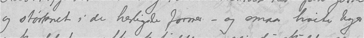og størknet i de herligste former – og smaa hvite byer
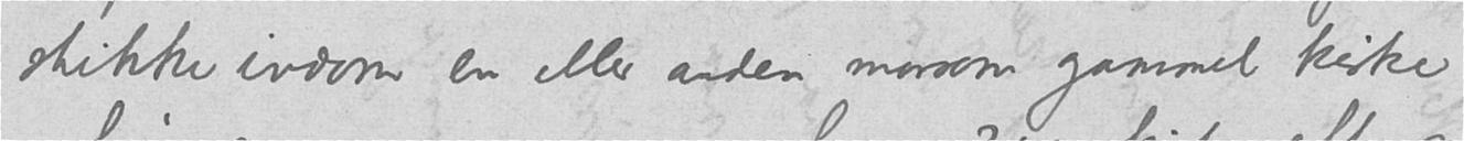stikke indom en eller anden morsom gammel kirke
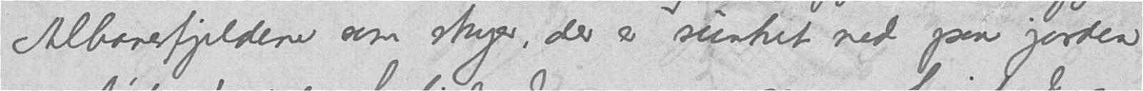Albanerfjeldene som skyer, der er sunket ned paa jorden
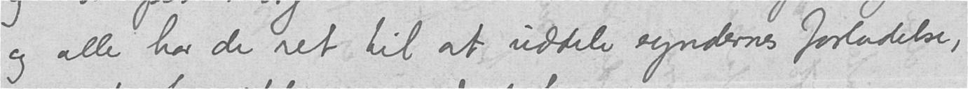og alle har de ret til at uddele syndernes forladelse,
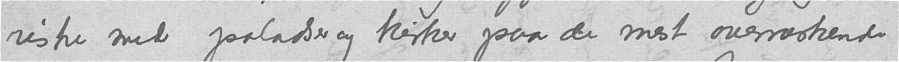riske med paladser og kirker paa de mest overraskende
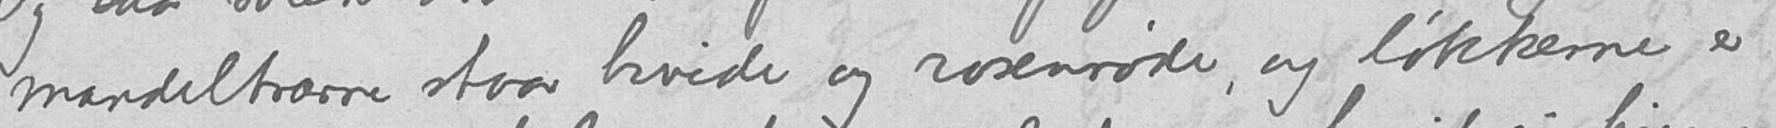mandeltrærne staar hvide og rosenrøde, og løkkerne er
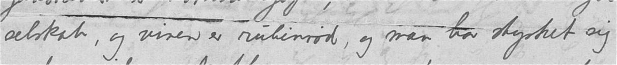selskab, og vinen er rubinrød, og man har styrket sig
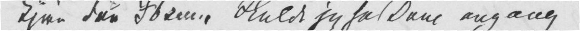Kjere Fru Ibsen. Skulde jeg se Dem engang
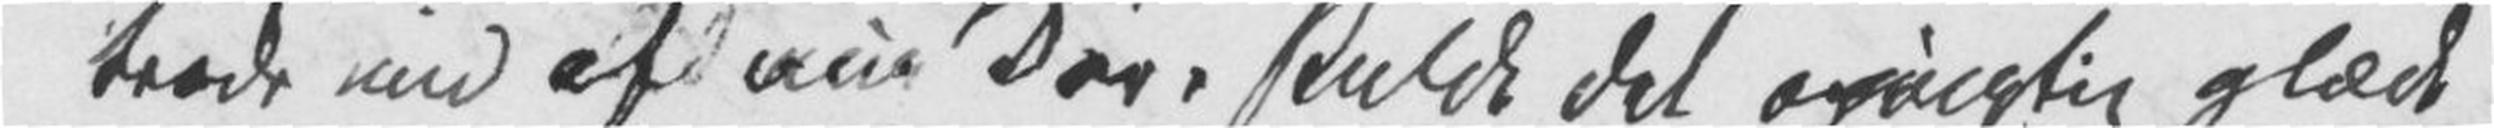træde ind af min Dør, skulde det oprigtig glæde
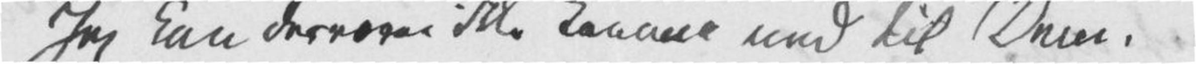Jeg kan desværre ikke komme ned til Dem,
